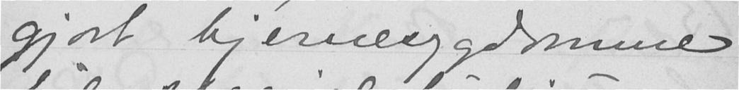gjort hjernesygdommer
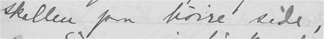skallen paa høire side,
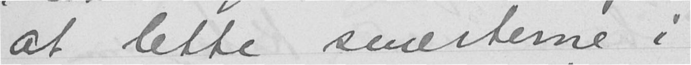at lette smerterne i
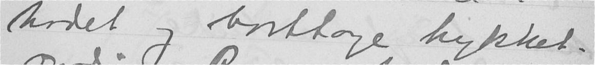hodet og borttage trykket.
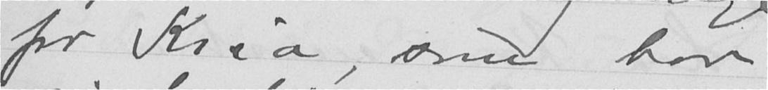fra Kria, som har
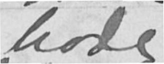hode
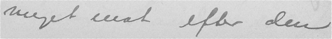meget mat efter den
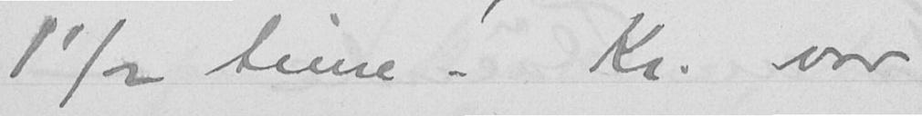1 ½ time. Kr. var
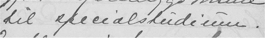til specialstudium.
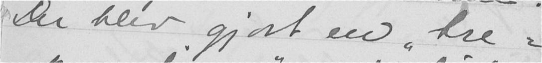Der blev gjort en "tre-
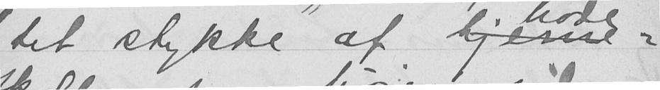tet stykke af hjerne-
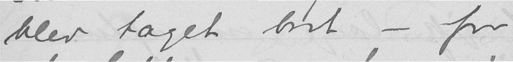blev taget bort - for
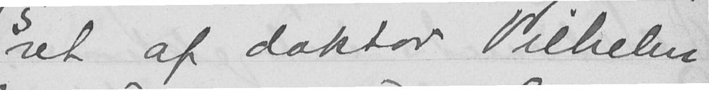ret af doktor Vilhelm
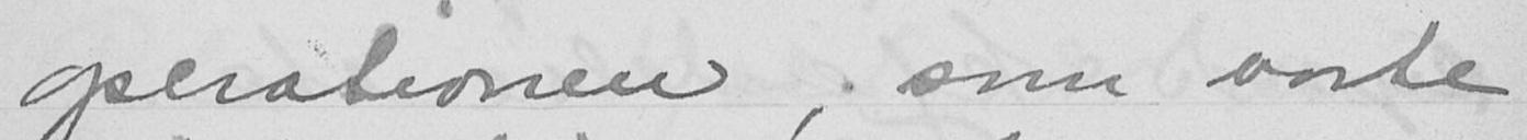operationen, som varte
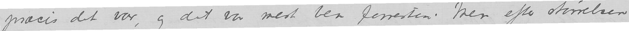præcis det var, og det var mest ben forresten. Men efter størrelsen
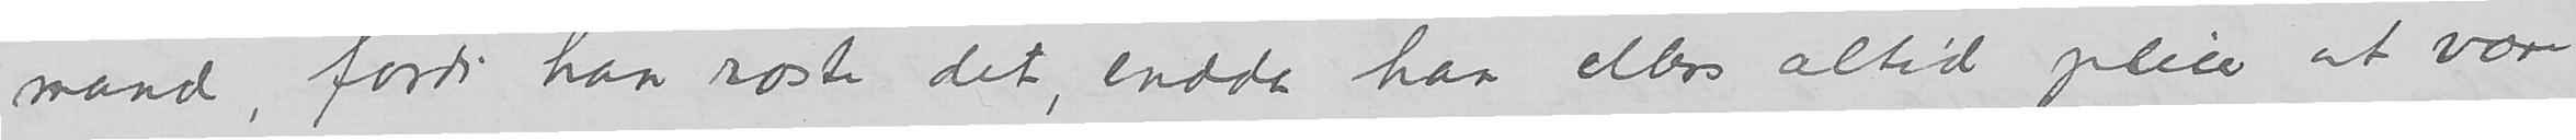mand, fordi han roste det, endda han ellers altid pleier at være
